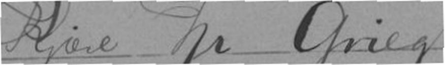Kjære hr Grieg
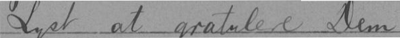Lyst at gratulere Dem,
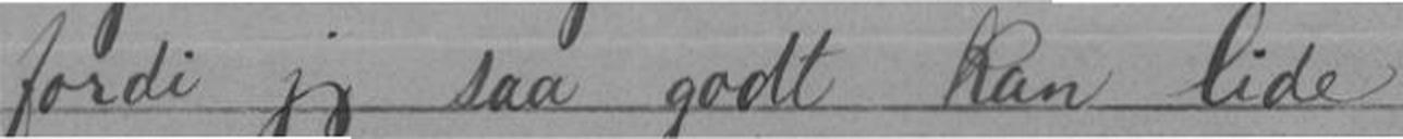fordi jeg saa godt kan lide
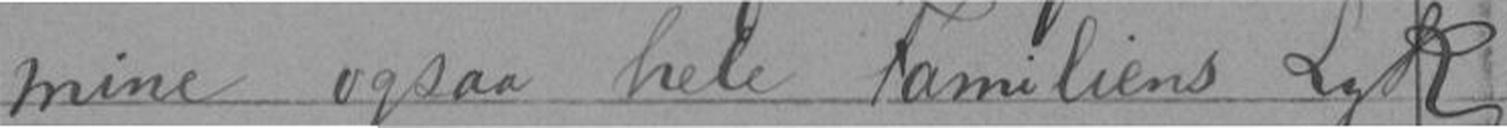mine ogsaa hele Familiens Lyk-
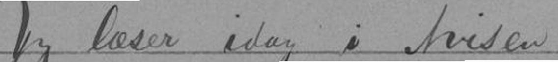Jeg læser idag i Avisen
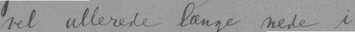vel allerede længe nede i
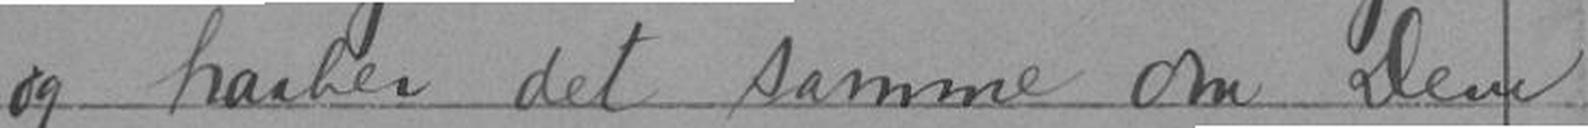og haaber det samme om Dem
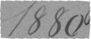1880
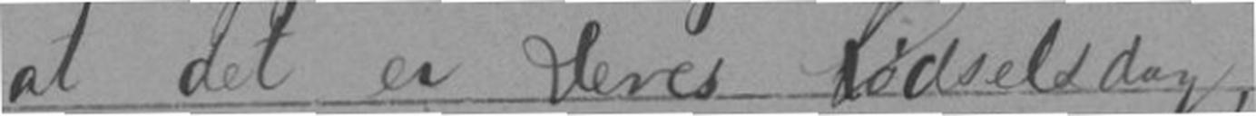at det er Deres Fødselsdag,
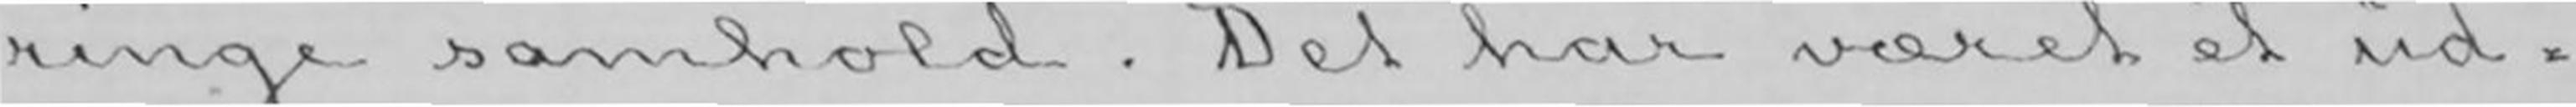ringe samhold. Det har været et ud-
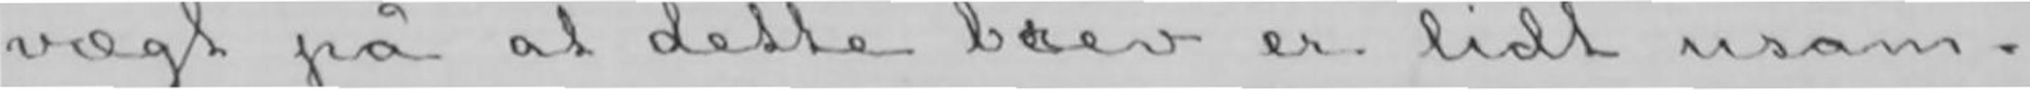vægt på at dette berev er lidt usam-
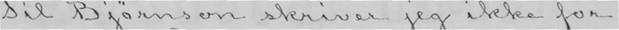Til Bjørnson skriver jeg ikke for
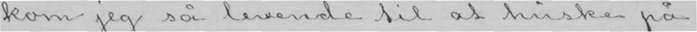kom jeg så levende til at huske på,
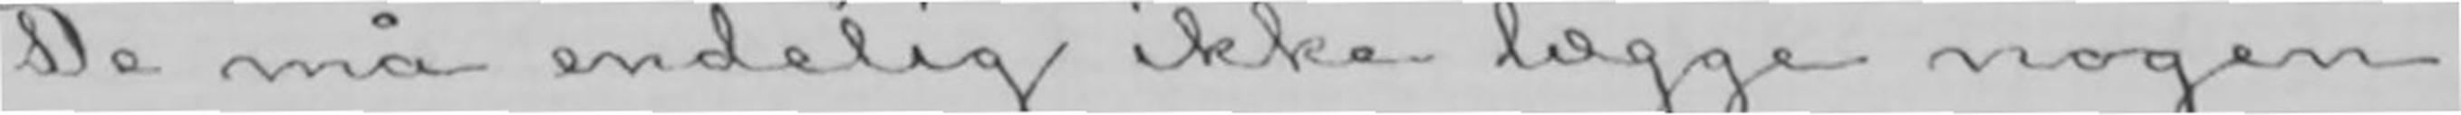De må endelig ikke lægge nogen
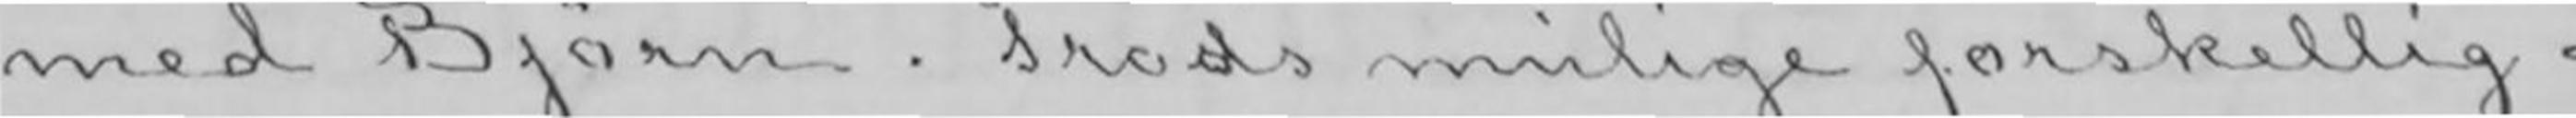med Bjørn. Trods mulige forskellig-
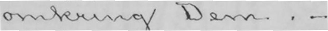omkring Dem.-
